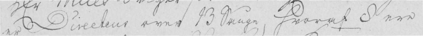er Directeur over 13 Sauge, hvoraf 8 ere
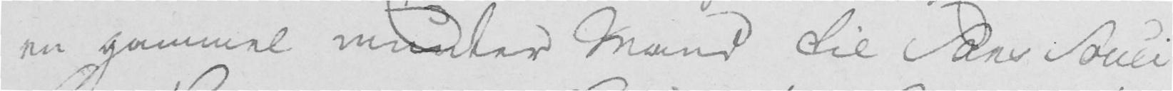en gammel munter Mand til Sans Souci
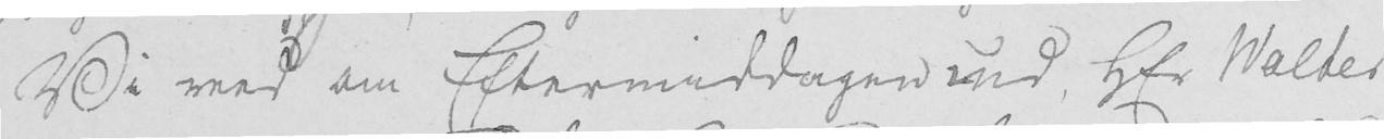Vi reed om Eftermiddagen ud, Hr Walter
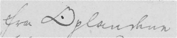fra Oplandene
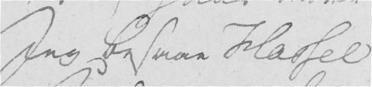Jeg besaae Hassel
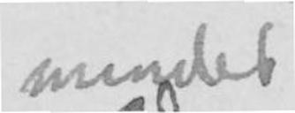mindes
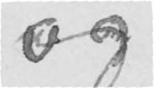og
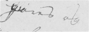giøres og
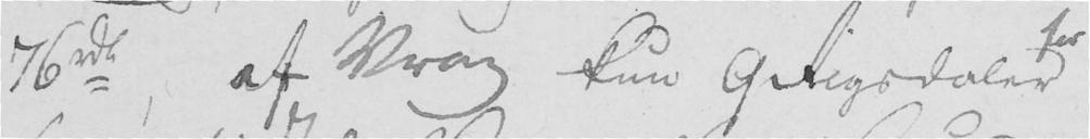76 rde af Vrag kun 9 Rigsdaler
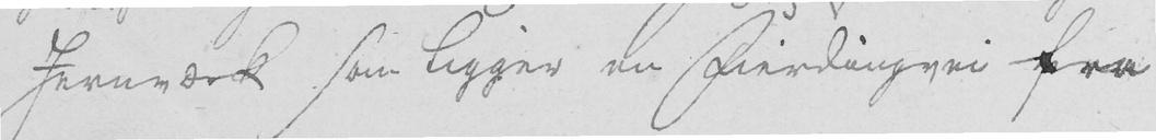Jernværk som ligger en Fierdingvei fra
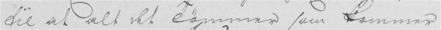til at alt det Tømmer som kommer
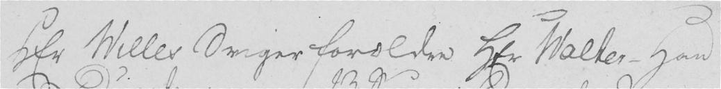Hr Willes Sviger forældre Hr Walter - Hand
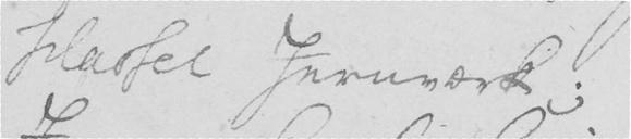Hassel Jernværk;
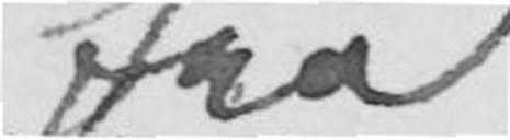fra
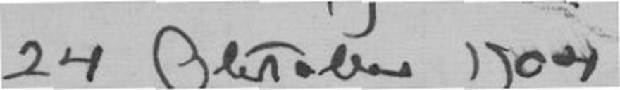24 Oktober 1904
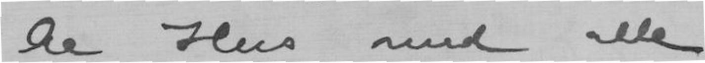ke Hus med alle
